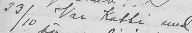23/10 Var Katti med
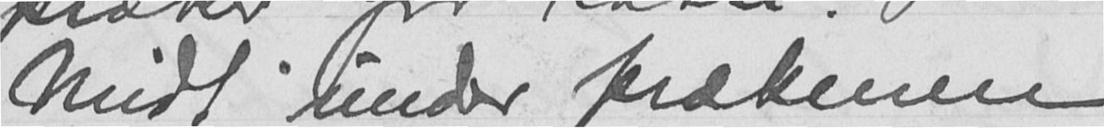Midt under prækenen
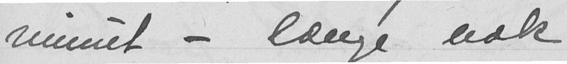minut - længe nok
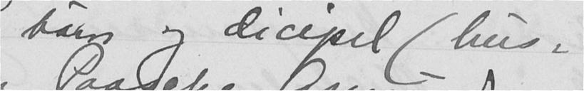børn og dicipel (hus-
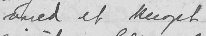vared et knapt
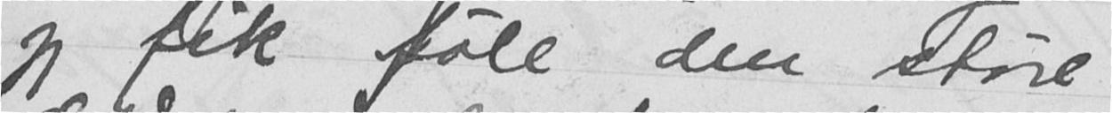jeg fik føle den store
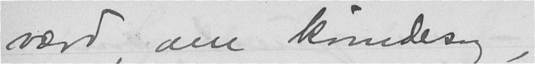værd, om kvindesag,
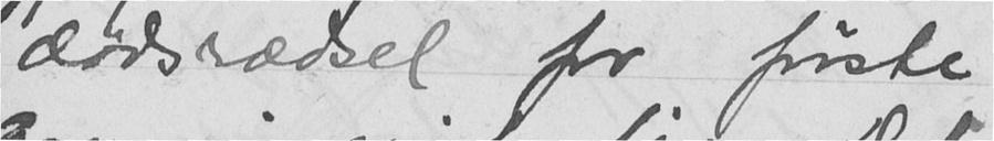dødsrædsel for første
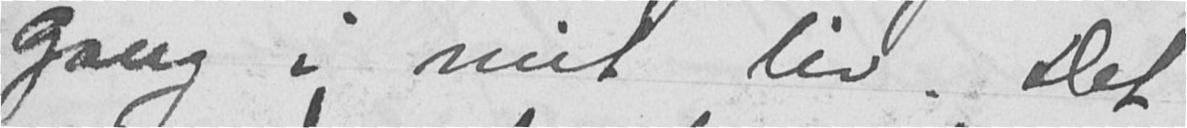gang i mit liv. Det
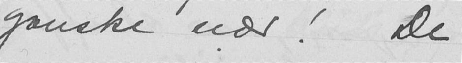ganske nær! De
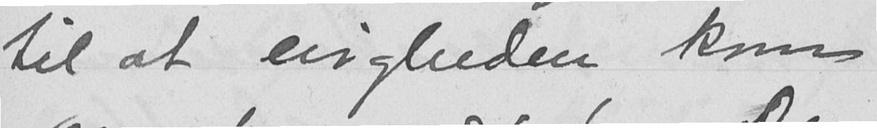til at evigheden kom
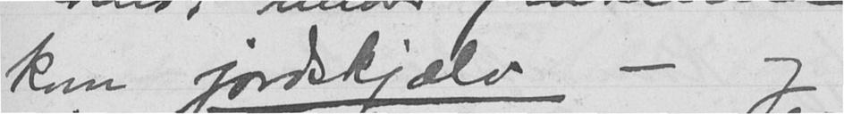kom jordskjælv - og
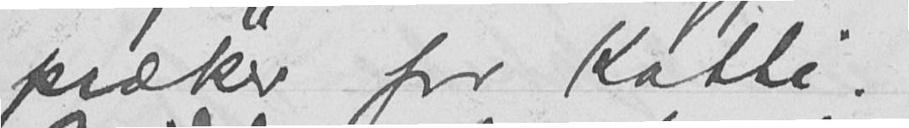præker for Katti.
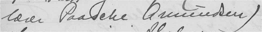lærer Paasche Amundsen)
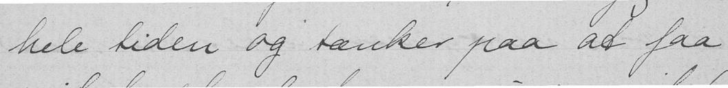hele tiden og tænker paa at faa
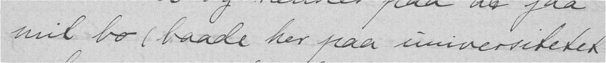mit bo (baade her paa universitetet
